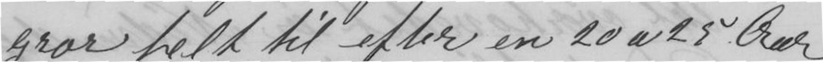gror helt til efter en 20 - 25 Aar
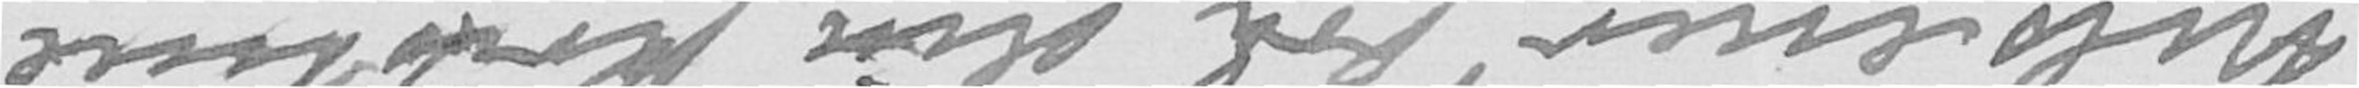hilsener fra din Kristine
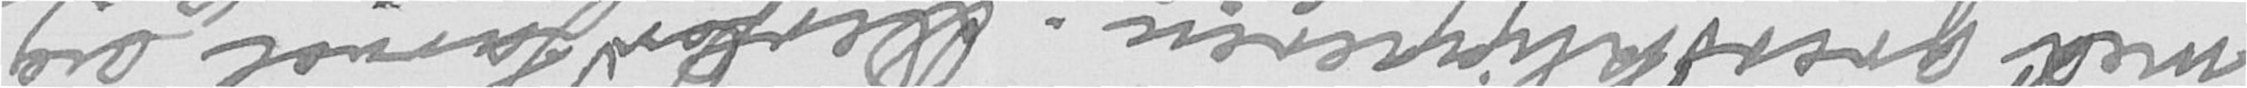med gressklipperen. Derfor farvel og
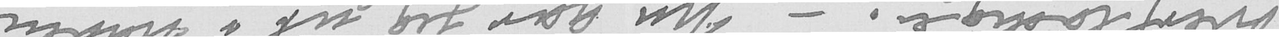overflødige. - Nu går jeg ut i haven
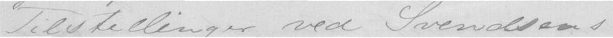Tilstellinger ved Svendsens
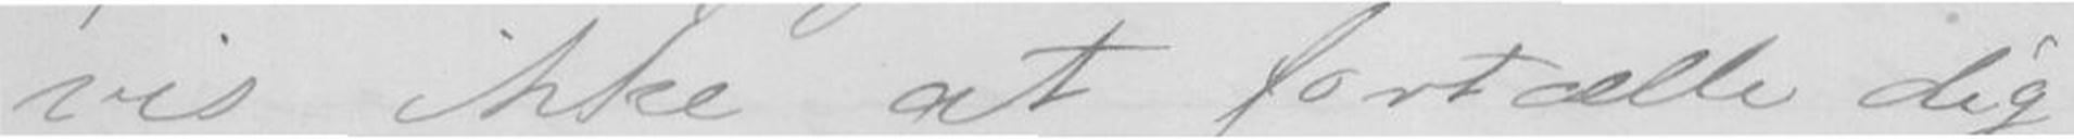vis ikke at fortælle dig
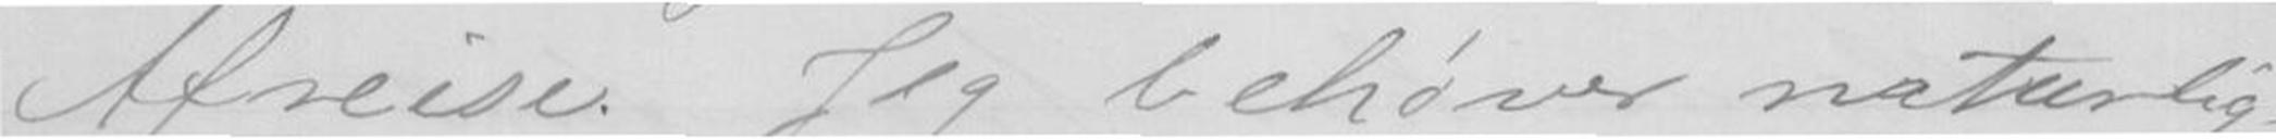Afreise Jeg behøver naturlig-
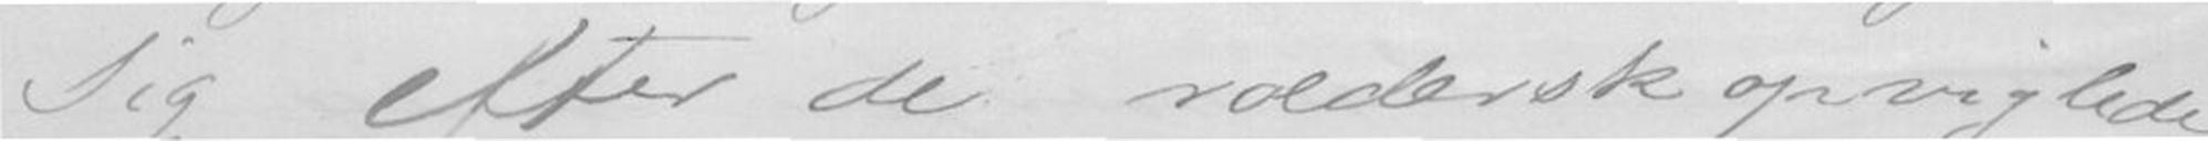sig efter de voldersk opviglede
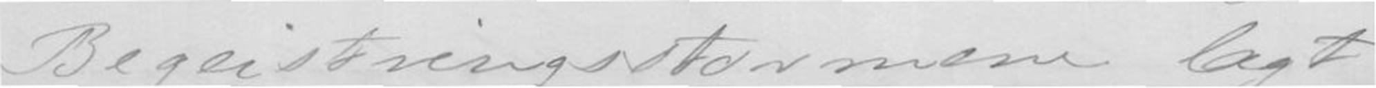Begeistringsstormen lagt
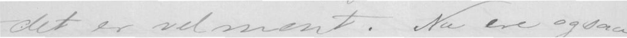- det er velment. Nu ere ogsaa
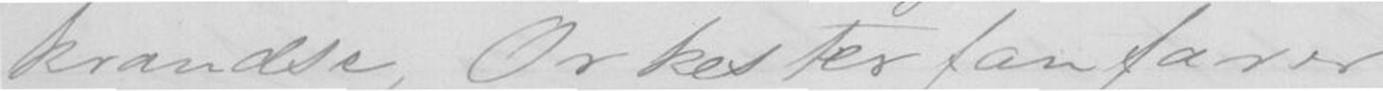krandse, Orkesterfanfarer
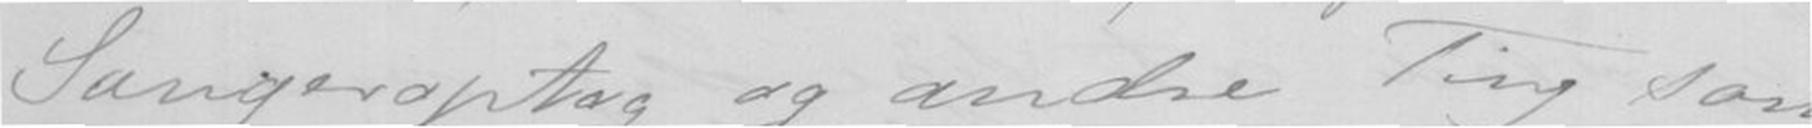Sangeropdrag og andre Ting som
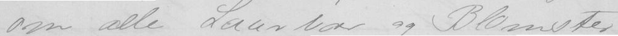om alle Laurbær og Blomster
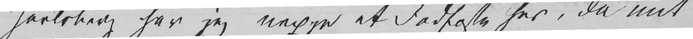Jarlsberg har jeg neppe et Fodfæste her, da mit
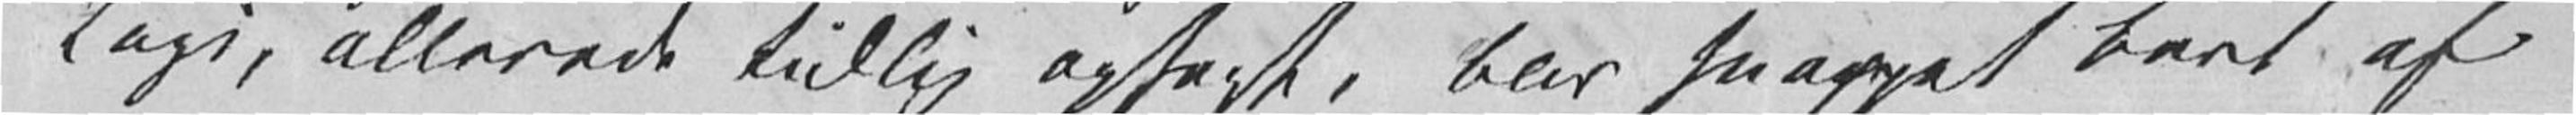Logi, allerede tidlig opsagt, blev snappet bort af
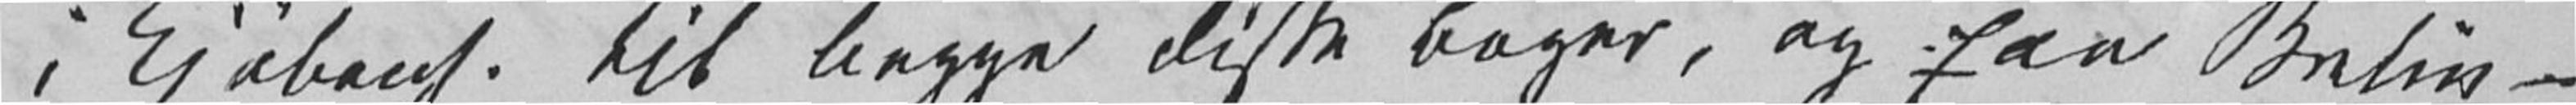i Kjøbenh. til begge disse Bøger, og paa Betin
